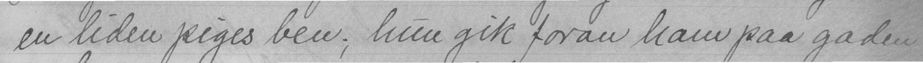en liden piges ben; hun gik foran ham paa gaden
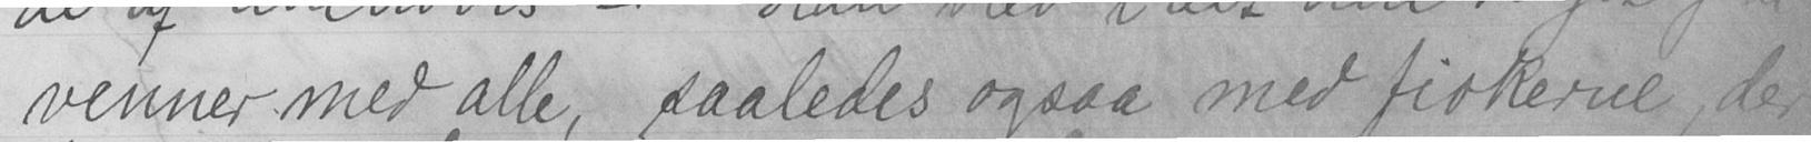venner med alle, saaledes ogsaa med fiskerne, der
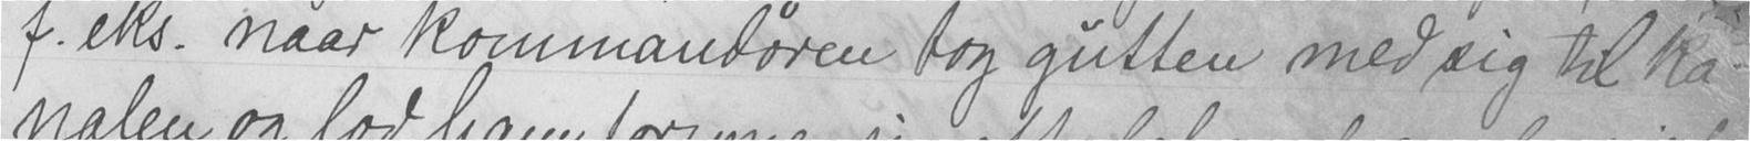f. eks. naar kommandøren tog gutten med sig til ka-
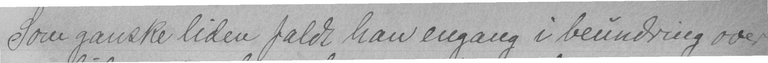Som ganske liden faldt han engang i beundring over
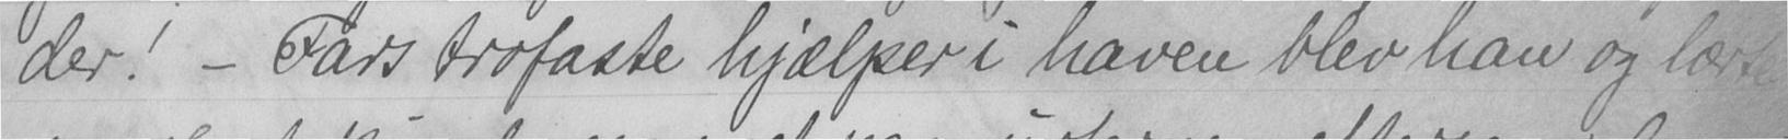der! - Fars trofaste hjælper i haven blev han og lærte
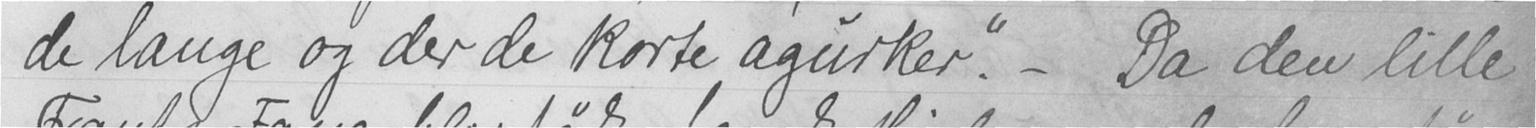de lange og der de korte agurker." - Da den lille
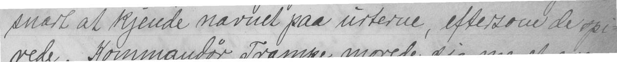snart at kjende navnet paa urterne, eftersom de spi-
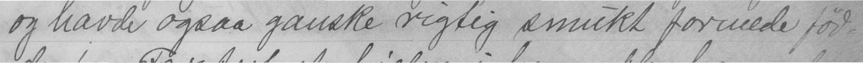og havde ogsaa ganske rigtig smukt formede fød-
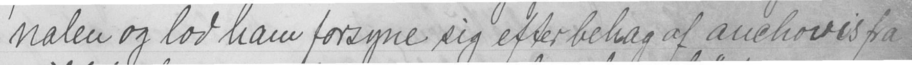nalen og lod ham forsyne sig efter behagaf anchovis fra
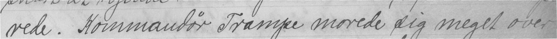rede. Kommandør Trampe morede sig meget over
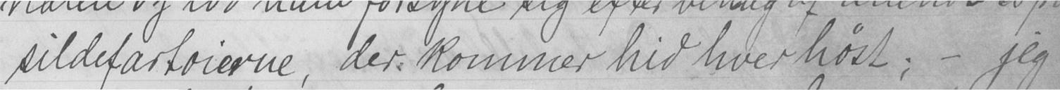sildefartøierne, der kommer hid hver høst; - jeg
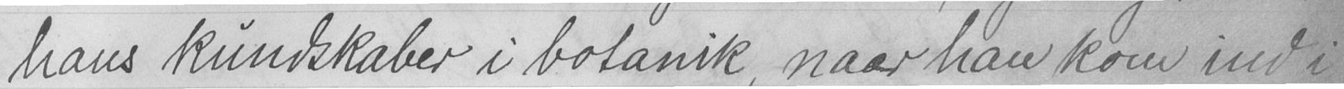hans kundskaber i botanik, naar han kom ind i
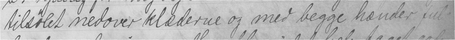tilsølet nedover klæderne og med begge hænder ful-
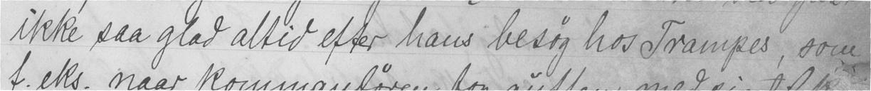ikke saa glad altid efter hans besøg hos Trampes, som
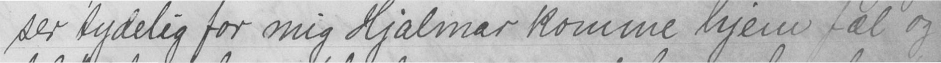ser tydelig for mig Hjalmar komme hjem fæl og
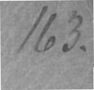163.
